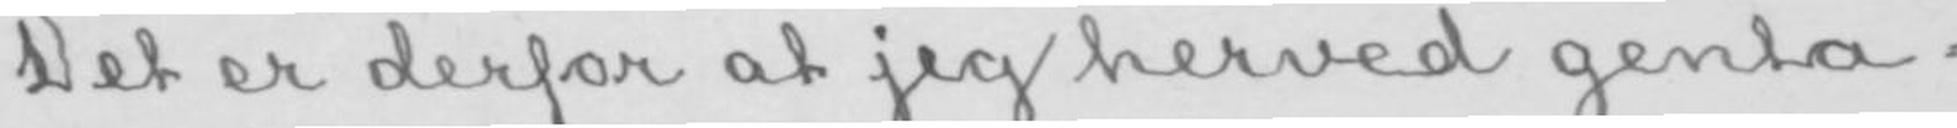Det er derfor at jeg herved genta-
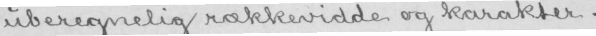uberegnelig rækkevidde og karakter.
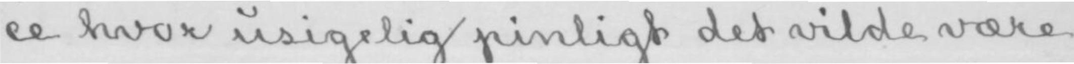ce hvor usigelig pinligt det vilde være
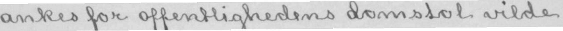ankes for offentlighedens domstol vilde
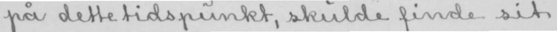på dette tidspunkt, skulde finde sit
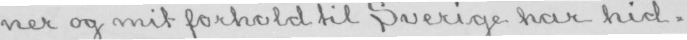ner og mit forhold til Sverige har hid-
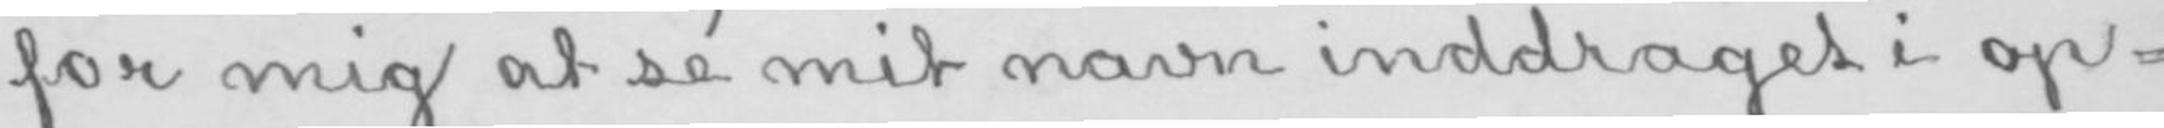for mig at sé mit navn inddraget i op-
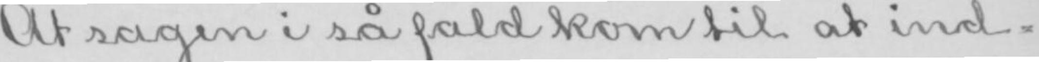At sagen i så fald kom til at ind-
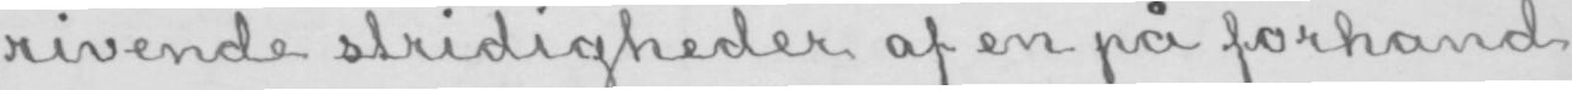rivende stridigheder af en på forhånd
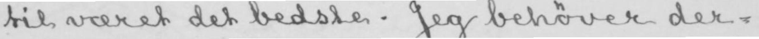til været det bedste. Jeg behøver der-
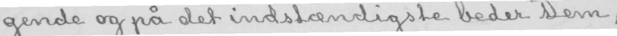gende og på det indstændigste beder Dem,
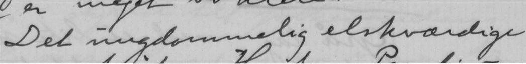Det ungdommelig elskværdige
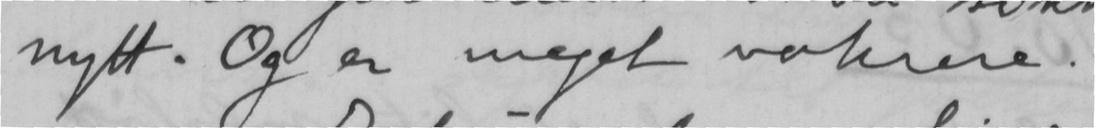nytt. Og er meget vakrere
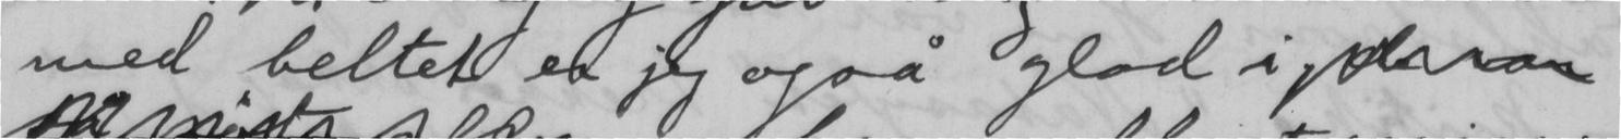med beltet er jeg også glad i
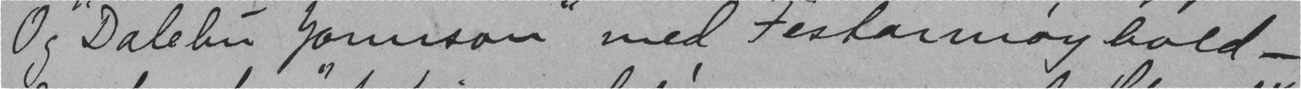Og "Dalebu Jonson med Festarmøy bold-
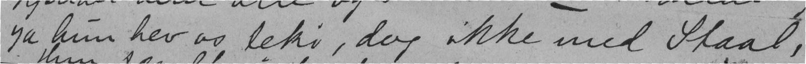Ja hun hev os teki, dog ikke med Staal,
